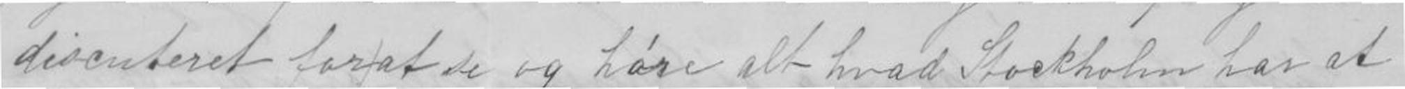discuteret barfat de og høre alt hvad Stockholm har at
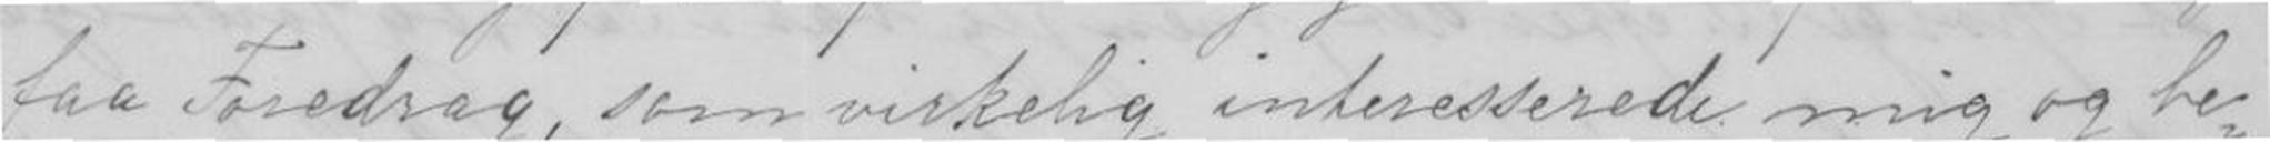faa Foredrag, som virkelig interesserede mig og be-
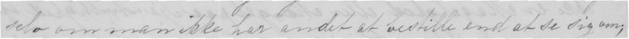selv om man ikke har andet at bestille end at se sig om,
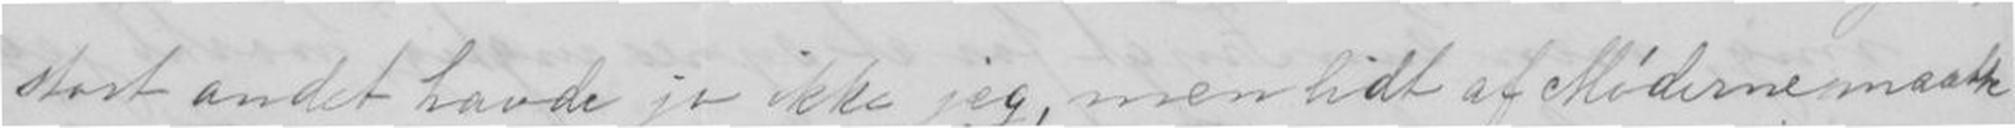stort andet havde jo ikke jeg, men Lidt af Møderme maatte
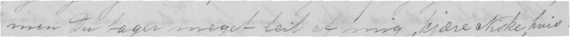men Du tager meget feil af mig, kjære Niske, hvis
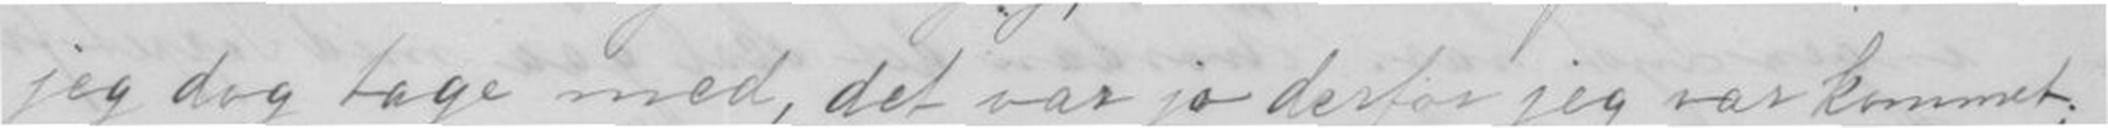jeg dog tage med, det var jo derfor jeg var kommet,
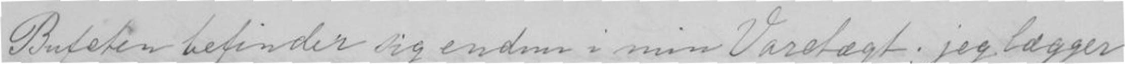Bufeten befinder sig endnu i min Varetægt; jeg lægger
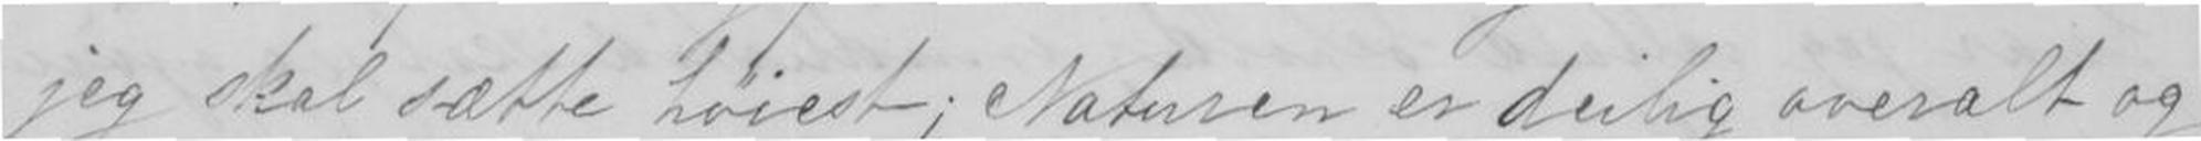jeg skal sætte høiest; Naturen er deilig overalt og
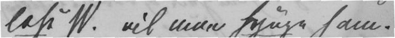loss W. vil man spuge ham.
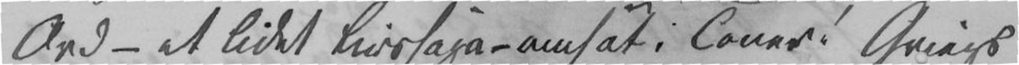Ord - et lidet Livahaja - omsat i Toner! Griegs
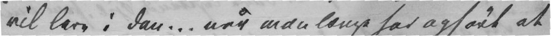vil leve i dan... når man længe har ophørt at
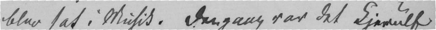sat i Musik. Dengang var det Kjerulf
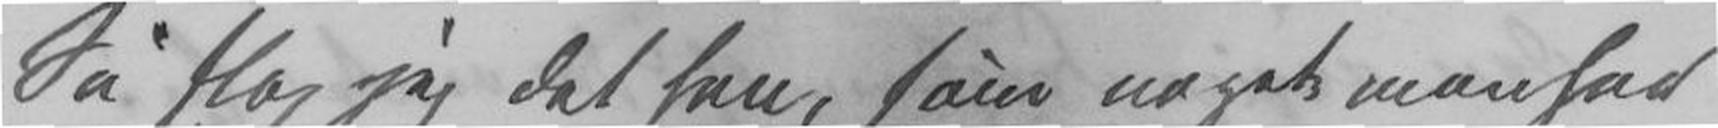Så flog jeg det han, som noget manhad
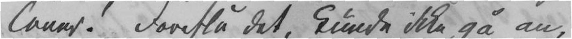Toner! Foreslå det, kunde ikke gå an.
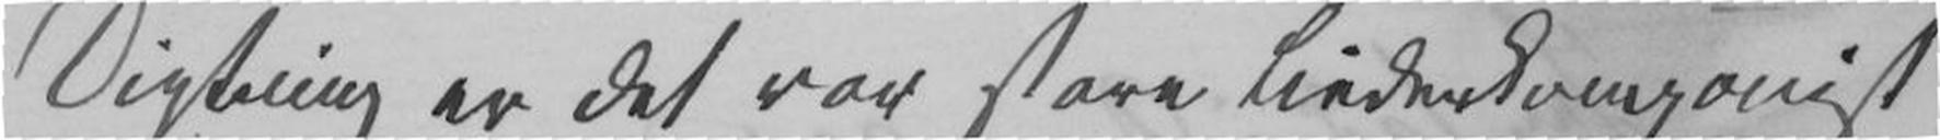Digtning er det vor store Liederkomponist
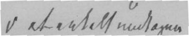i et antall undtagen
